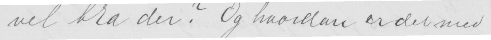vel bra der? Og hvordan er det med
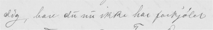dig, bare du nu ikke har forkjølet
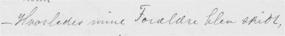– Hvorledes mine Forældre blev skilt,
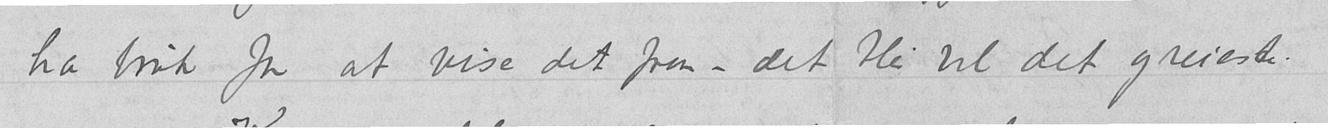ha bruk for at vise det frem – det blir vel det greieste.
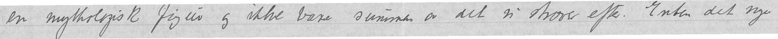en mythologisk figur og ikke bare summen av det vi stræver efter. Enten det nye
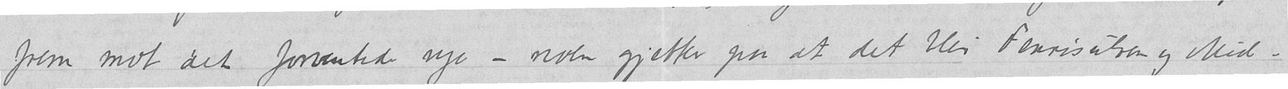frem mot det forventede nye – noen gjetter paa at det blir Fenrisulren og Mid-
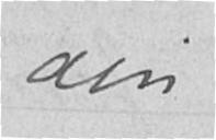din
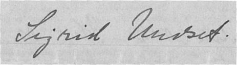Sigrid Undset.
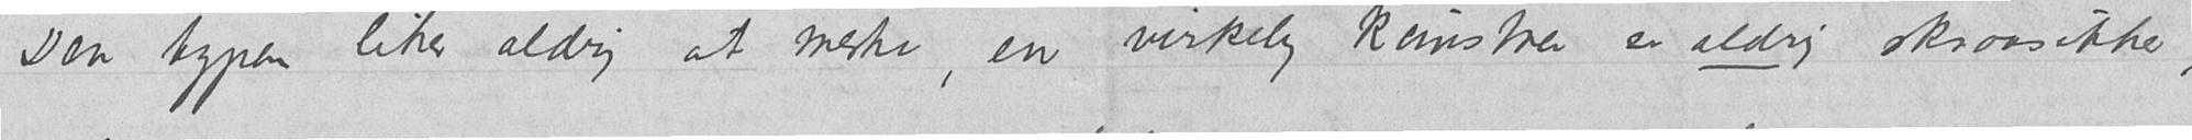Den typen liker aldrig at merke, en virkelig kunstner er aldrig skraasikker,
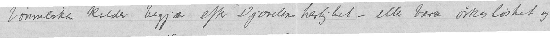bønneboken kalder begjær efter Djævelens herlighet – eller bare ørkesløshet og
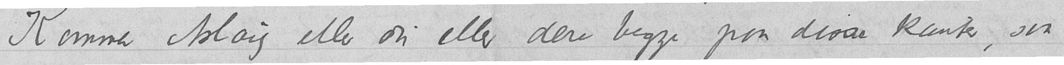Kommer Åslaug eller du eller dere begge paa disse kanter, saa
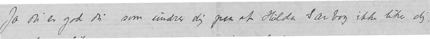Ja du er god du som undrer dig paa at Hulda Garborg ikke liker dig.
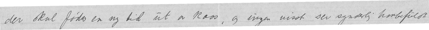der skal fødes en ny tid ut av kaos, og ingen visst ser synderlig haabefuldt
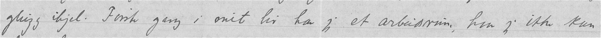glugg ihjel. Første gang i mit liv har jeg et arbeidsrum, hvor jeg ikke kan
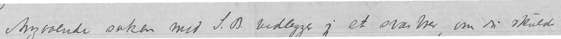Angaaende saken med S.B. vedlegger jeg et svarbrev, om du skulde
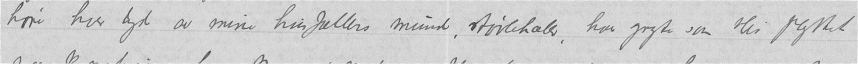høre hver lyd av mine husfællers mund, støvlehæler, hver gryte som blir flyttet
